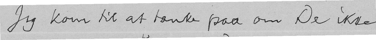Jeg kom til at tænke paa om De ikke
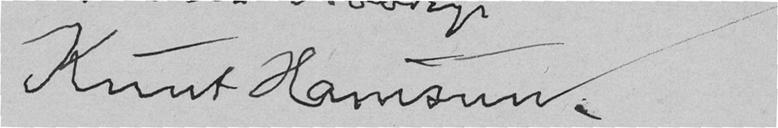Knut Hamsun.
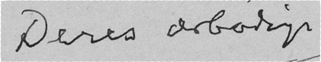Deres ærbødige
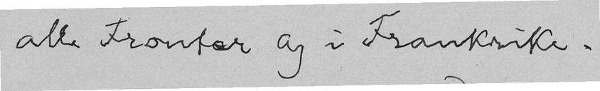alle Fronter og i Frankrike.
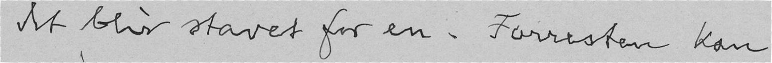det blir stavet for en. Forresten kan
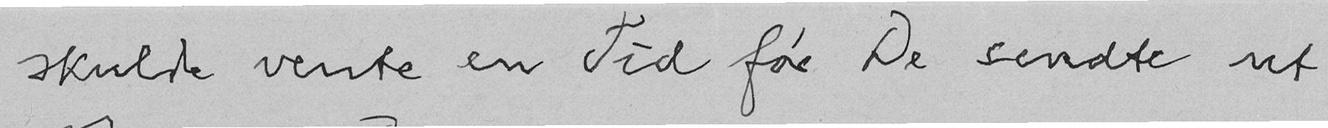skulde vente en Tid før De sendte ut
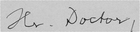Hr. Doctor,
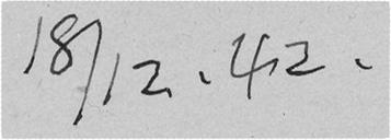18/12. 42.
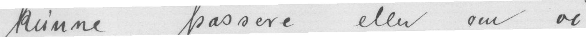kunne passere eller om vi
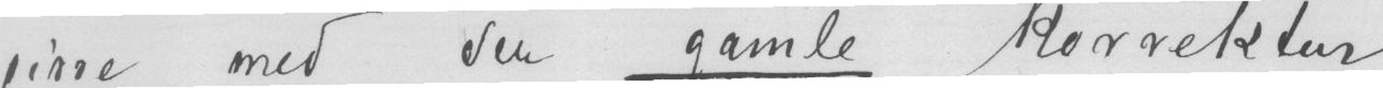disse med den gamle Korrektur
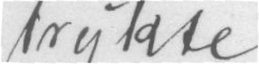trykte.
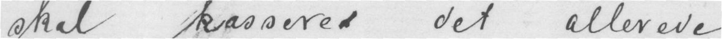skal kassere det allerede
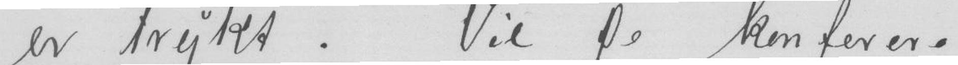"er trykt". Vil De konferere
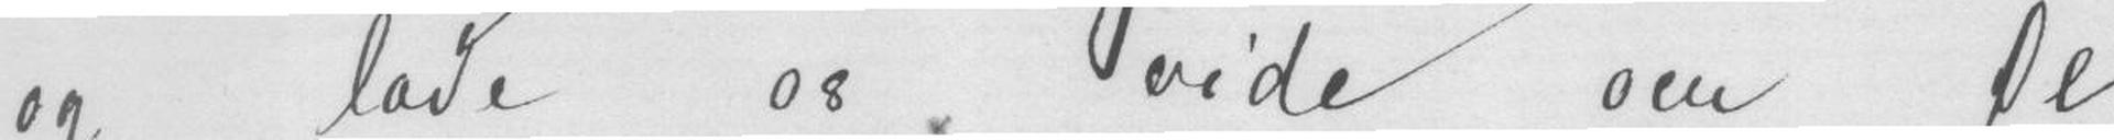og love os vide sen De
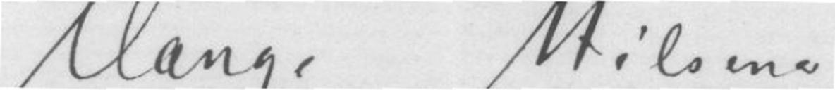Mange Hilsener fr.
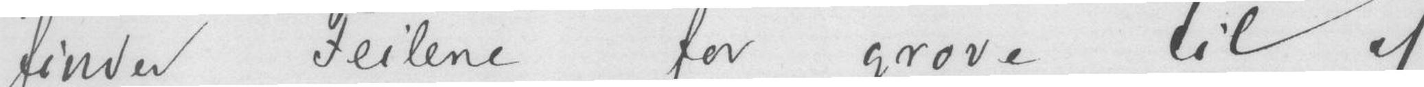finder Feilene for grove til af
